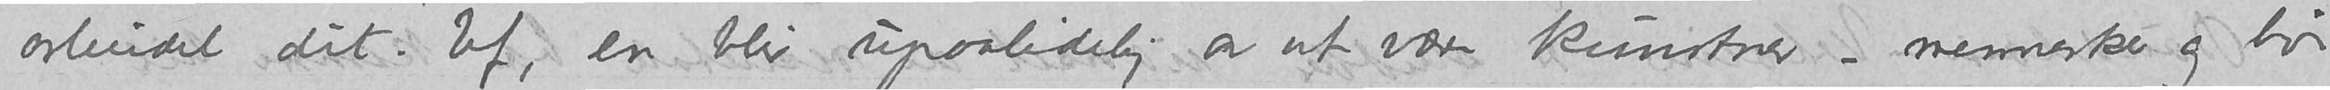arbeidet dit: Uf, en blir upaalidelig av at være kunstner – mennesker og liv
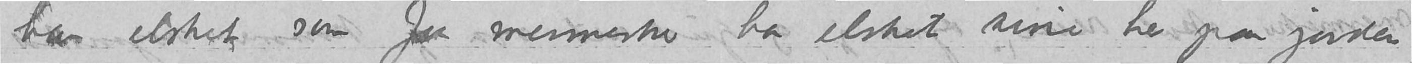han elsket som faa mennesker har elsket sine her paa jorden,
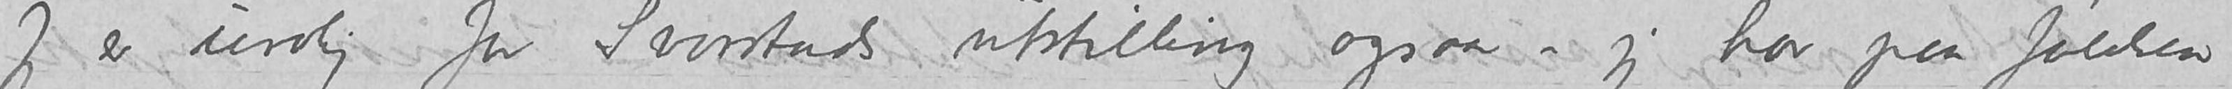–Jeg er urolig for Svarstads utstilling ogsaa – jeg har paa følelsen
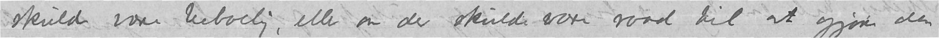skulde være beboelig, eller om der skulde være raad til at gjøre den
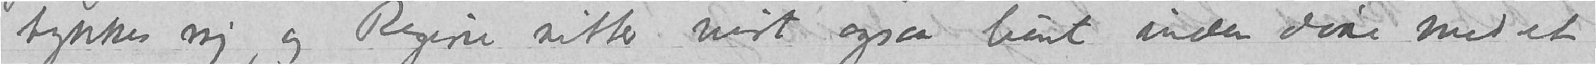tykkes mig, og Regine sitter vist ogsaa lunt inden døre med et
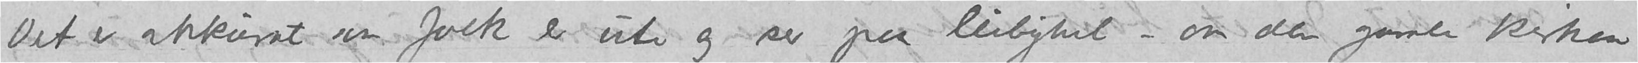Det er akkurat som folk er ute og ser paa leilighet – om den gamle kirken
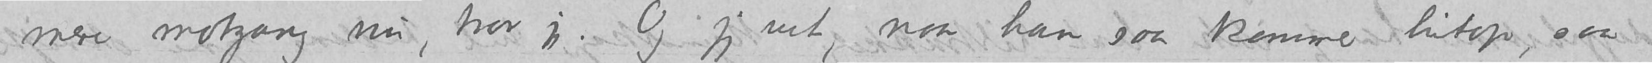mere motgang nu, tror jeg. Og jeg vet, naar han saa kommer hitop, saa
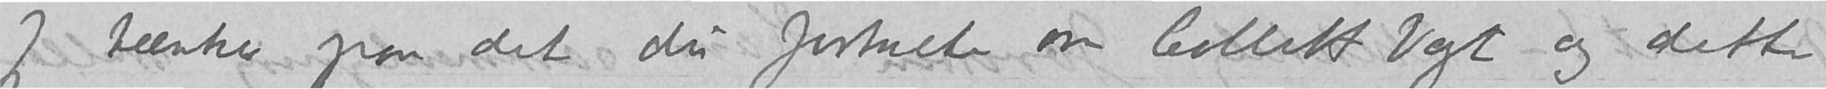–Jeg tænker paa det du fortalte om Collett Vogt og dette
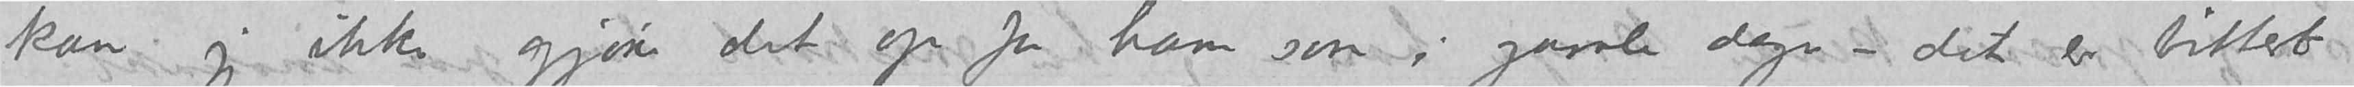kan jeg ikke gjøre det op for ham som i gamle dager – det er bittert
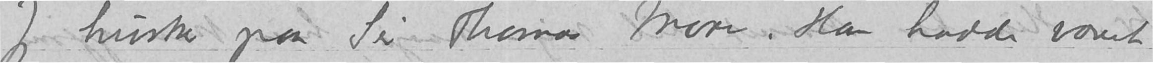Jeg husker paa Sir Thomas More. Han hadde været
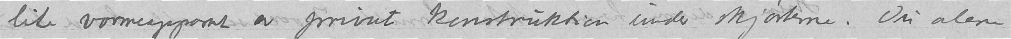lite varmeapparat av privat konstruktion under skjørterne. Du alene
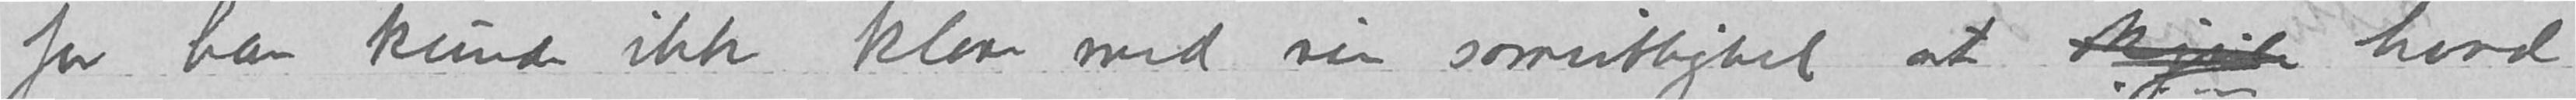for han kunde ikke klare med sin samvittighed at skjule hvad
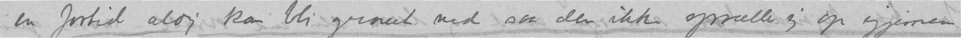en fortid aldri kan bli gravet ned saa den ikke spræller sig op igjennem
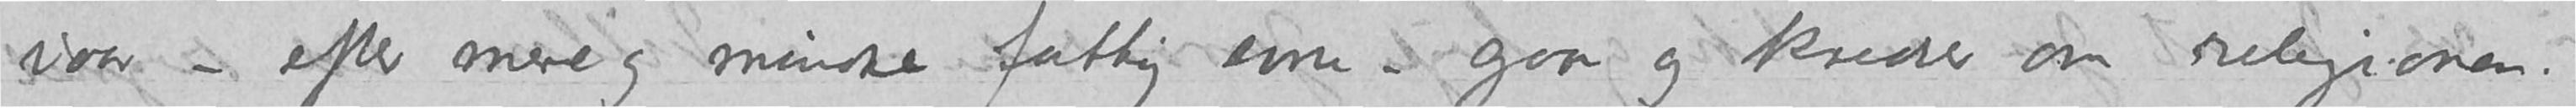iaar – efter mere og mindre fattig evne – gaar og kredser om religionen.
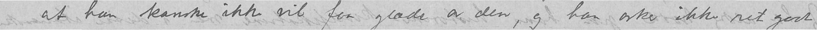at han kanske ikke vil faa glæde av den, og han orker ikke ret godt
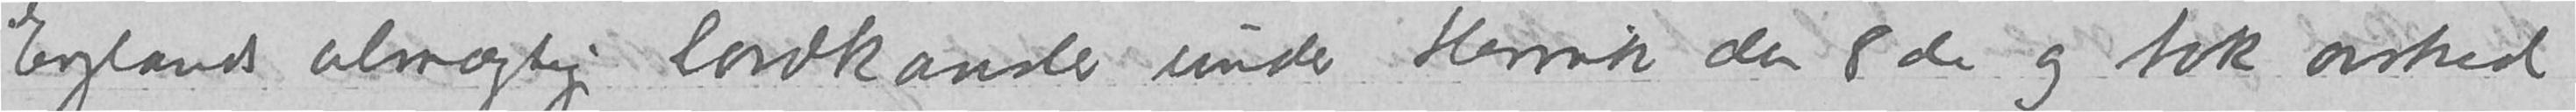Englands almægtige lordkansler under Henrik den 8de og tok avsked
